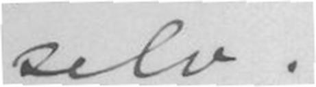selv.
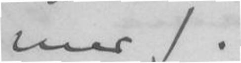mer).
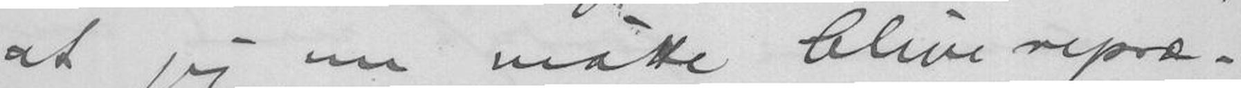at jeg nu måtte blive repræ-
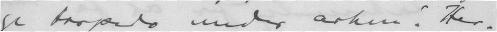ge tropedo under arken! Her-
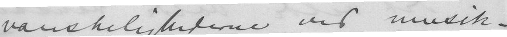vanskelighederne ved musik-
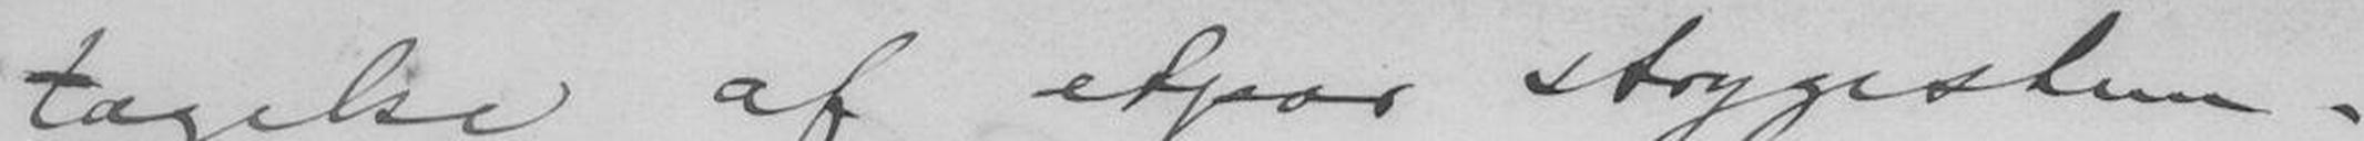tagelse af etpar strygestem-
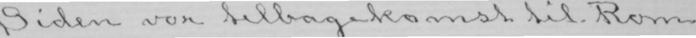Siden vor tilbagekomst til Rom
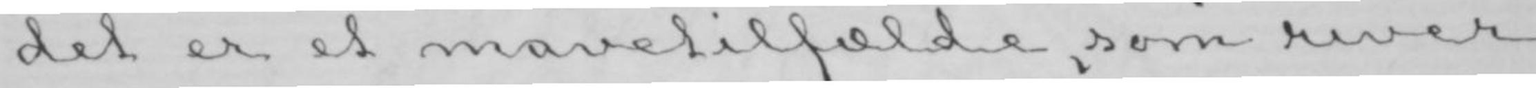det er et mavetilfælde, som river
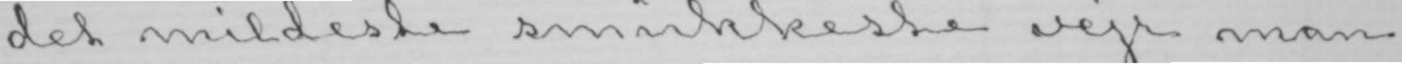det mildeste smukkeste vejr man
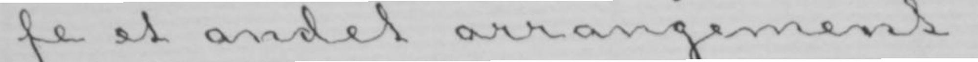fe et andet arrangement.
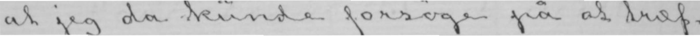at jeg da kunde forsøge på at træf-
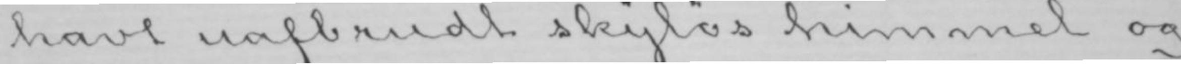havt uafbrudt skyløs himmel og
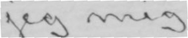jeg mig
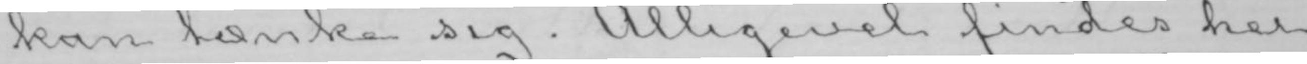kan tænke sig. Alligevel findes her
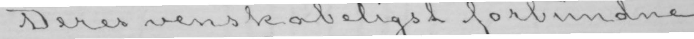Deres venskabeligst forbundne
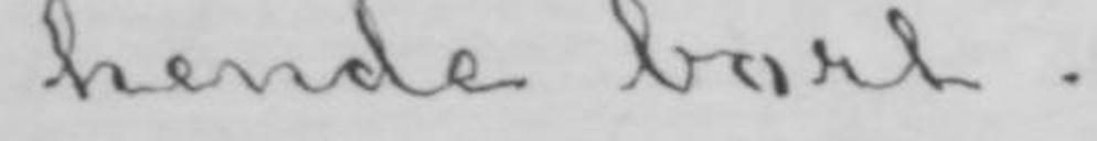hende bort.
